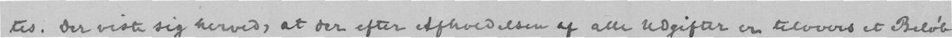tes. Der viste sig herved, at der efter Afholdelsen af alle Udgifter er tilovers et Beløb
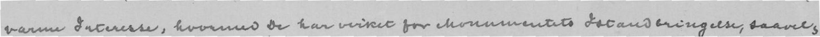varme Interesse, hvormed De har virket for Monumentets Istandbringelse, saavelsom
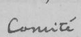Comité
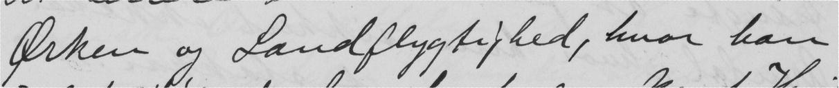Ørken og Landflygtighed, hvor han
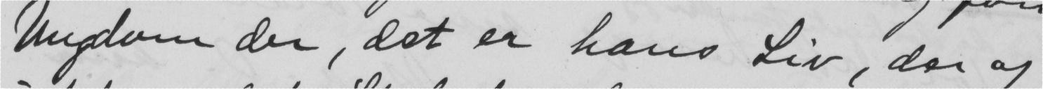Ungdom der, det er hans Liv, der og
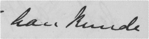han kunde
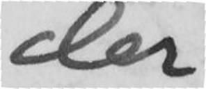der
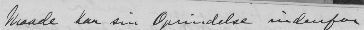Maade kan sin Oprindelse indenfor
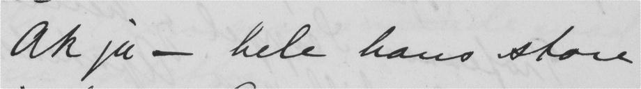Ak ja - hele hans store
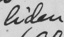liden
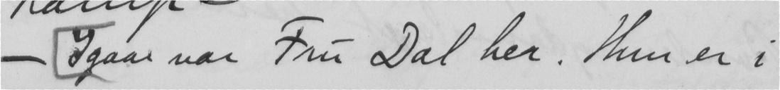- Igaar var Fru Dal her. Hun er i
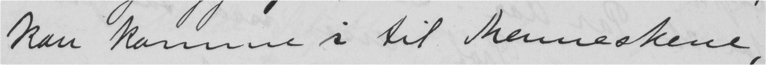kan komme i til Menneskene,
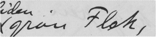grøn Flok,
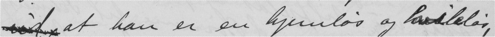at han er en hjemløs og hvileløs,
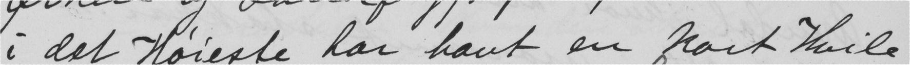det Høieste har havt en kort Hvile
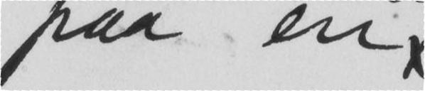paa en
